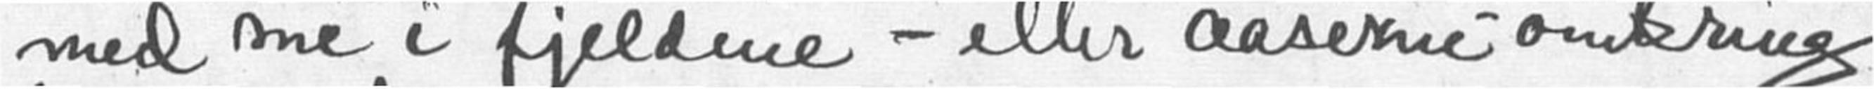med sne i fieldene - eller aaserne omkring
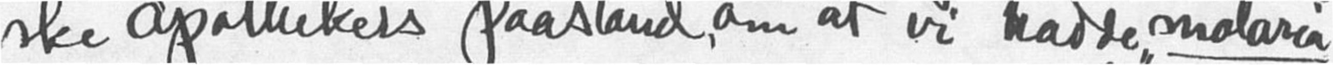ske apothekers paastand, om at vi hadde "malaria"
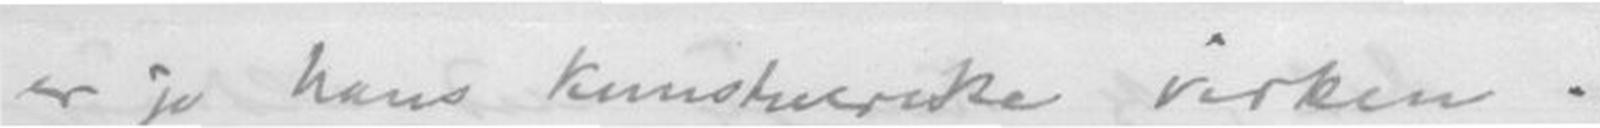er jo hans kunstnerske virken.
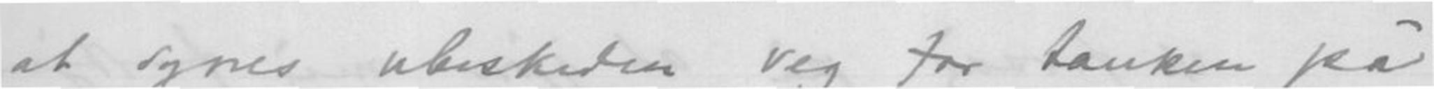at synes ubeskeden veg for tanken på
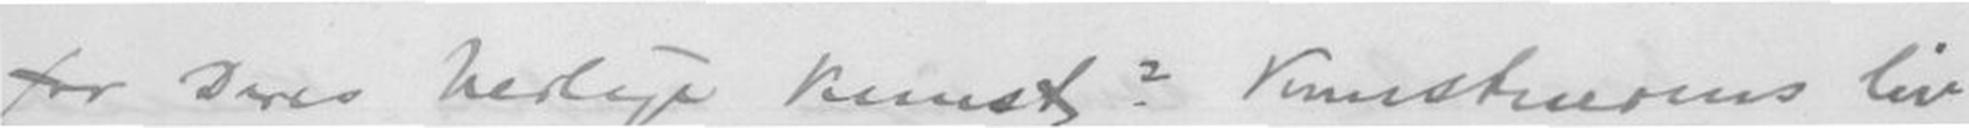for Deres herlige kunst? Kunstnerens liv
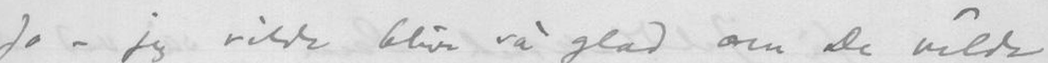Jo - jeg vilde blive så glad om De vilde
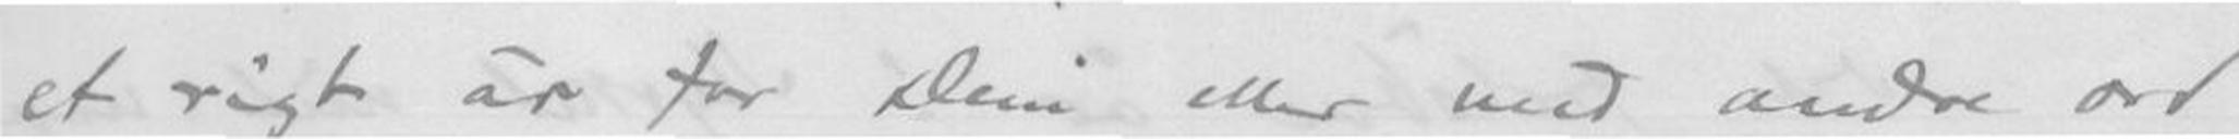et rigt år for Dem eller med andre ord
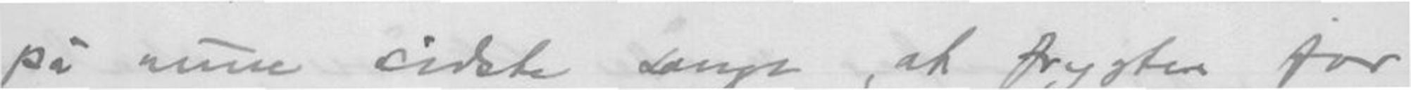på mine sidste sange, at frygten for
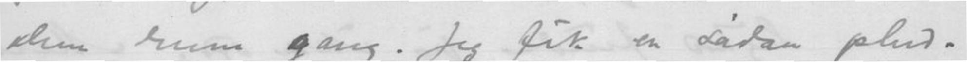Dem denne gang. Jeg fik en sådan plud-
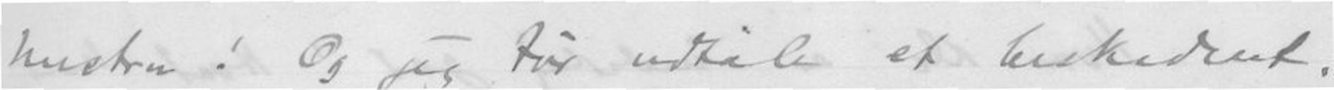hustru! Og jeg tør udtale et beskedent,
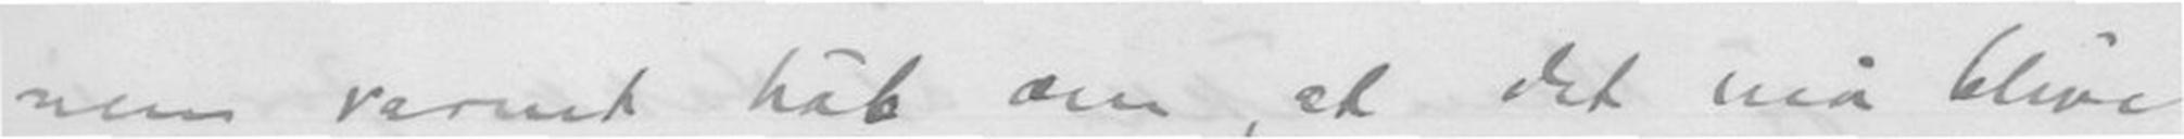men varmt håb om, at det må blive
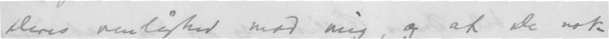Deres venlighed mod mig, og at De nok
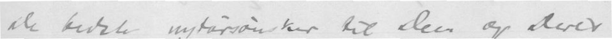De bedste nytårsønsker til Dem og Deres
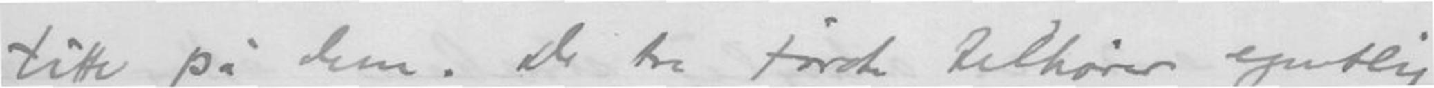titte på dem. De tre første tilhører egentlig
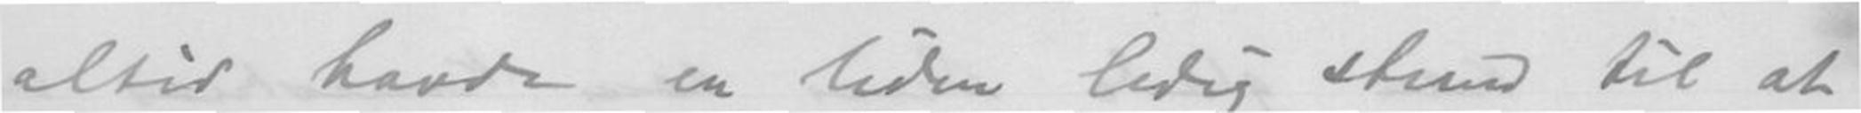altid havde en liden ledig stund til at
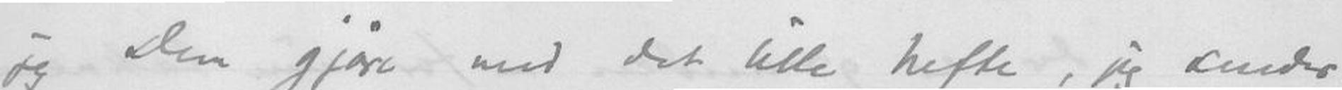jeg Dem gjøre med det lille heftet, jeg sender
